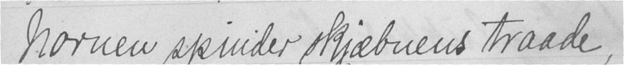Nornen spinder skjæbnens traade,
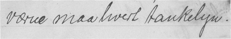værne maa hvert tankelyn.
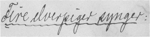Fire elverpiger synger.
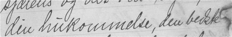din hukommelse, den bedste
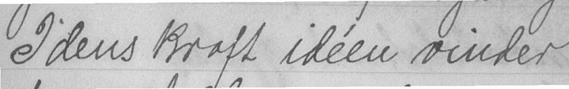I dens kraft idéen vinder
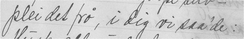plei det frø, i dig vi saa'de:
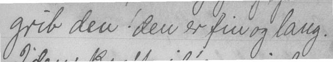grib den! den er fin og lang.
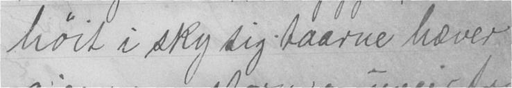høit i sky sig taarne hæver
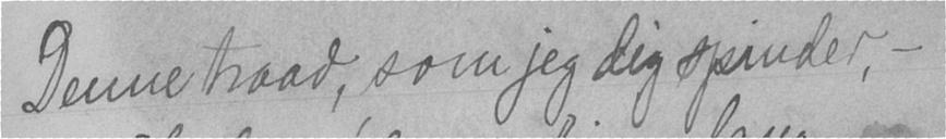Denne traad, som jeg dig spinder, -
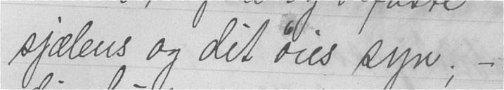sjælens og dit øies syn; -
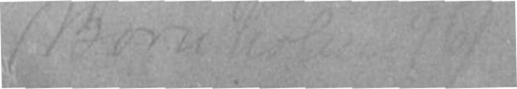(Bornholm 1896)
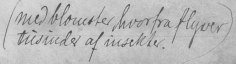(med blomster hvorfra flyver tusinder af insekter.)
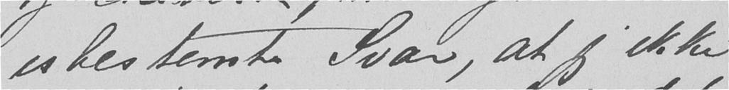ubestemte Svar, at jeg ikke
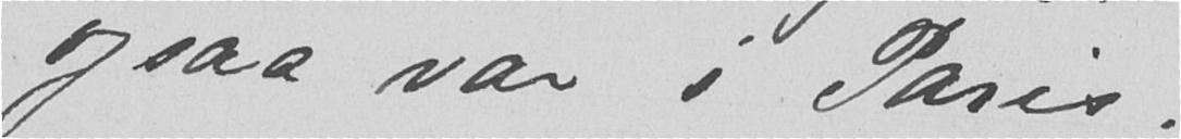ogsaa var i Paris.
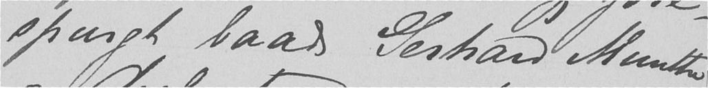spurgt baade Gerhard Munthe
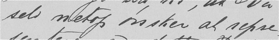selv netop ønsker at repre-
